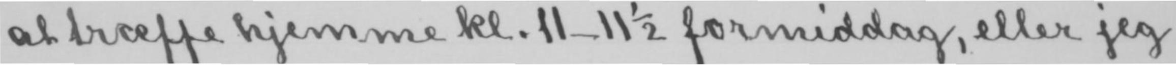at træffe hjemme kl. 11-11½ formiddag, eller jeg
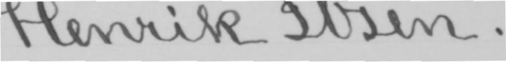Henrik Ibsen.
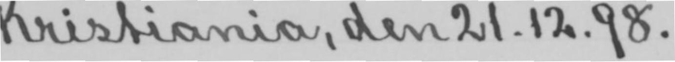Kristiania, den 21.12.98.
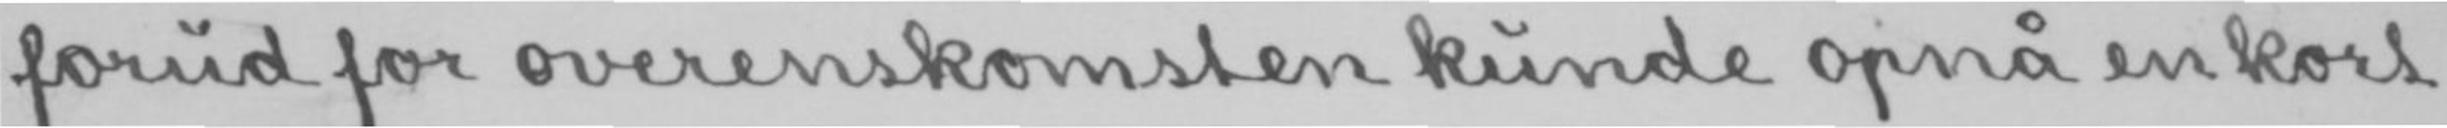forud for overenskomsten kunde opnå en kort
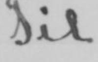Til
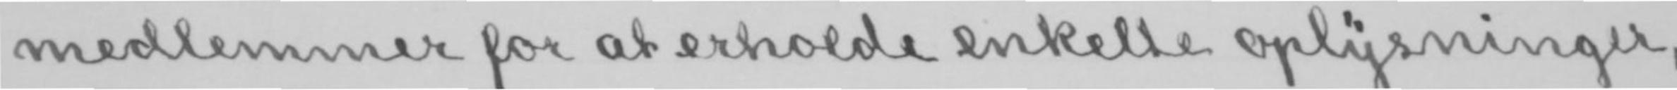medlemmer for at erholde enkelte oplysninger,
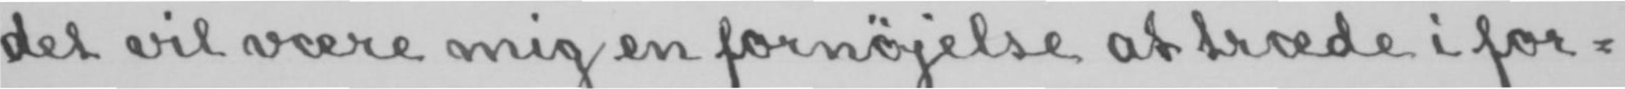det vil være mig en fornøjelse at træde i for-
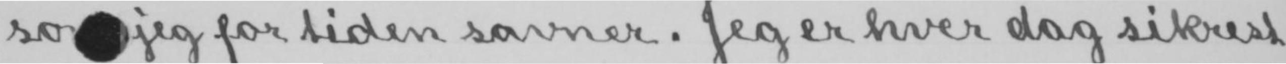so jeg for tiden savner. Jeg er hver dag sikrest
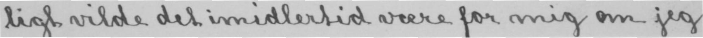ligt vilde det imidlertid være for mig om jeg
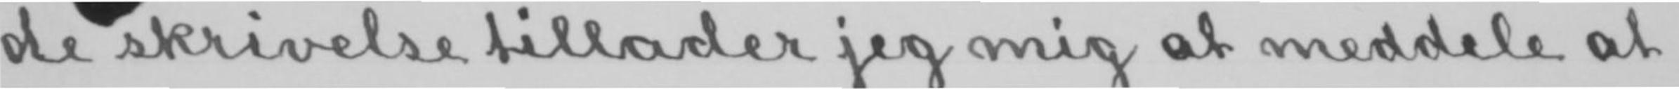de skrivelse tillader jeg mig at meddele at
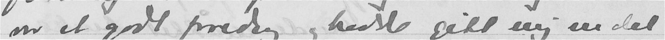var et godt foredrag og hadde gitt mig en del
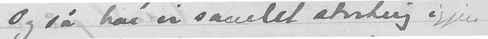Og så har vi samlet storting igjen,
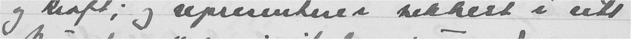og kraft; og representerer sikkert i sitt
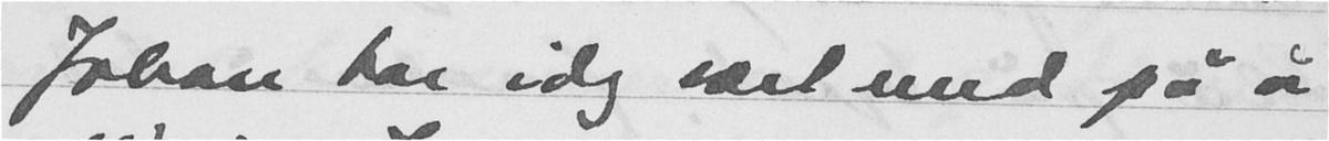Johan har idag vært med på å
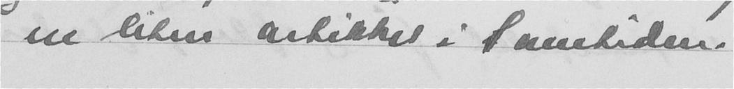en liten artikkel i Samtiden.
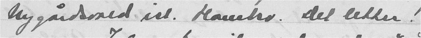Nygårdsvold ist. Hambro. Det letter!
